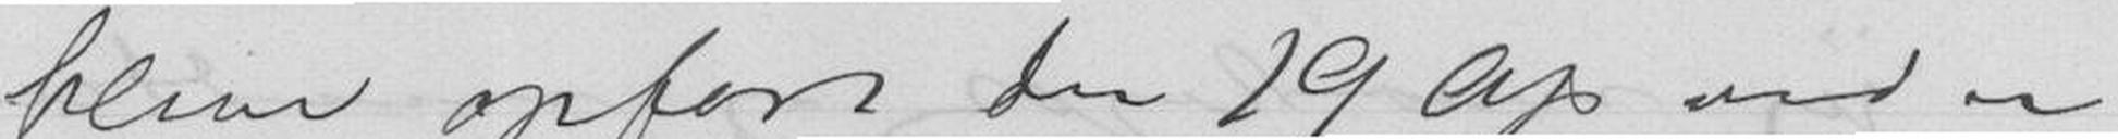blive opført den 19 Ap ved en
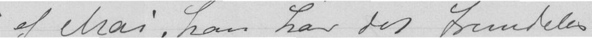af Mai, han har det fremdeles
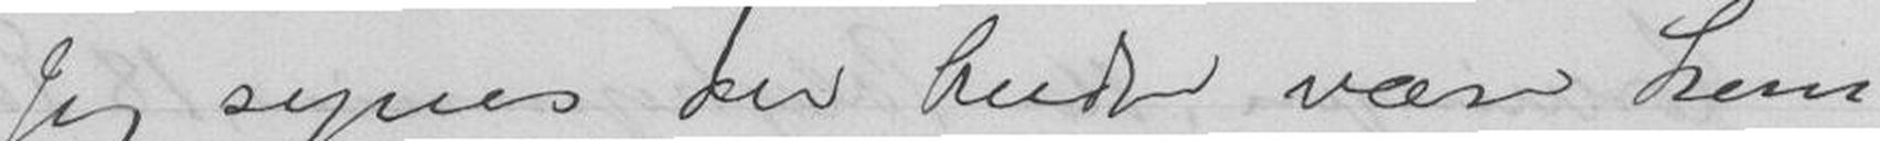Jeg synes du burde være kom
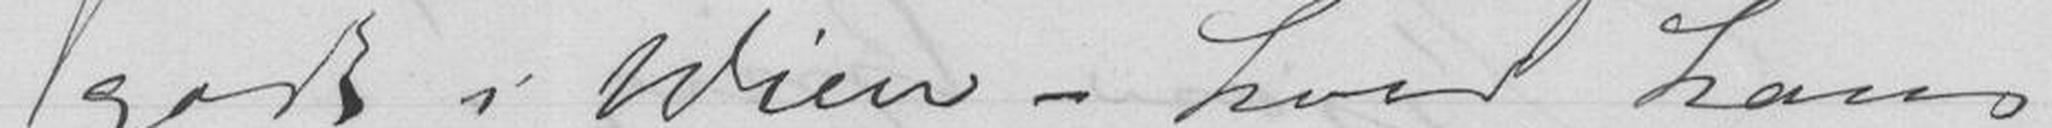godt i Wien - hvad hans
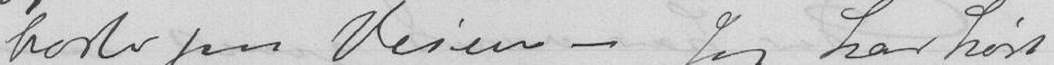borte paa Veien - Jeg har hørt
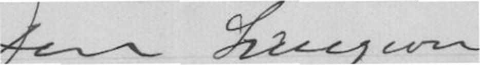Din hengivne
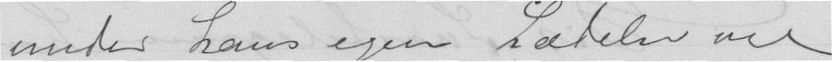under hans egen Lædelse vil
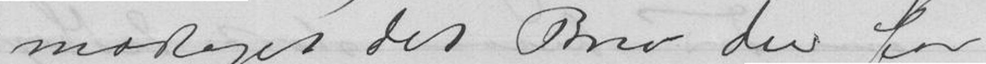modtaget det Brev du for
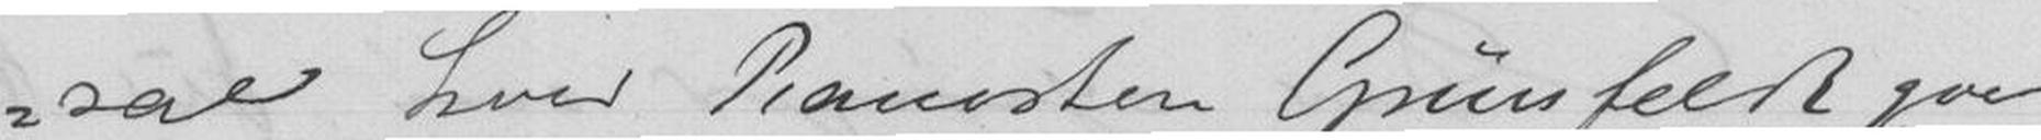sal hvor Pianisten Grünfeldt gav
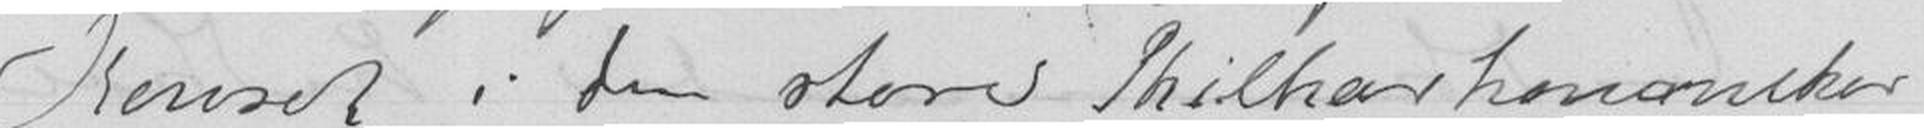Konsert i den store Philharhononeker
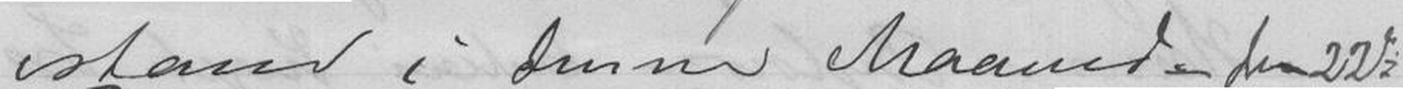istand i denne Maaneden - den 22e
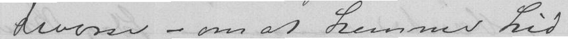diverse - om at komme hid
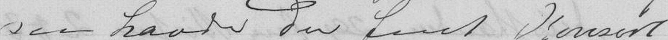saa havde Du faaet Konsert
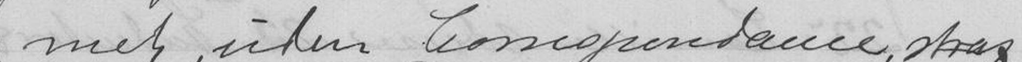met uden Correspondance, strax
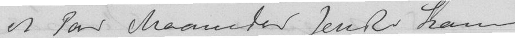et Par Maaneder sendte ham
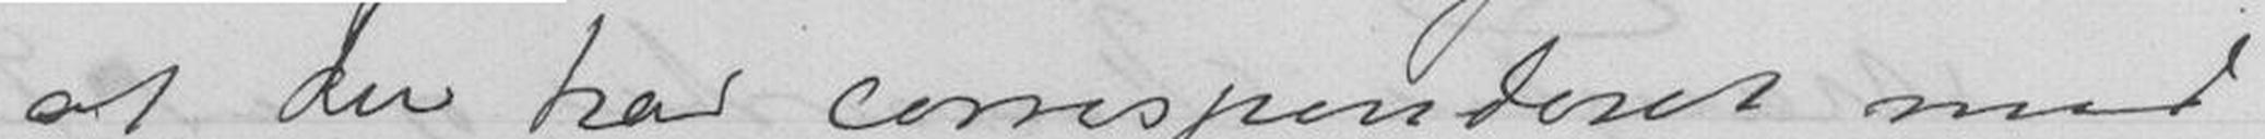at du har corresponderet med
